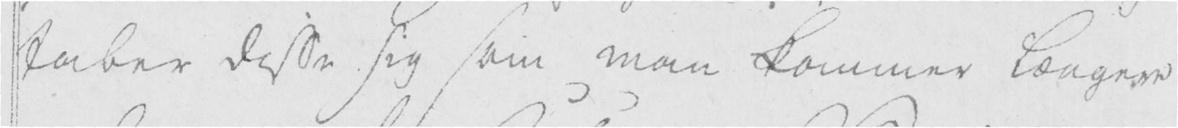taber disse sig som man kommer længere
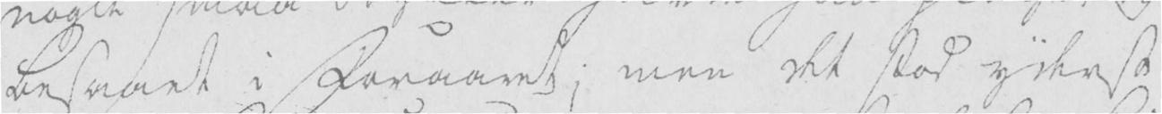besaaet i Foraaret; men det stod yderst
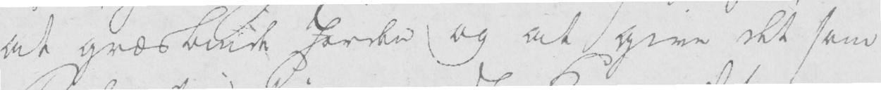at græsbinde Jorden og at give det som
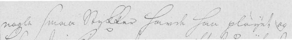nogle smaa Stykker havde han pløyet og
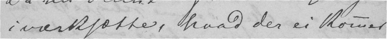iværksætte, hvad der ei kommer
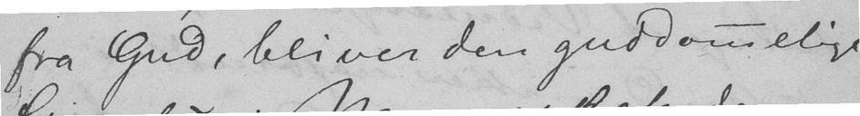fra Gud, bliver den guddommelige
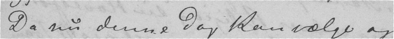Da nu denne dog kan vælge og
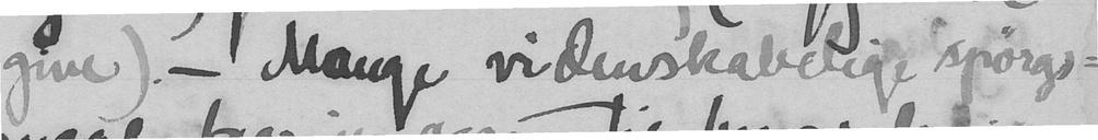give). - Mange videnskabelige spørgs-
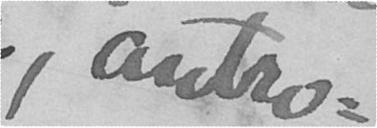, antro-
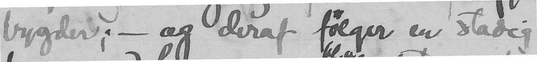bygder, - og deraf følger en stadig
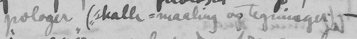pologer ("skalle-maaling og tegninger") -
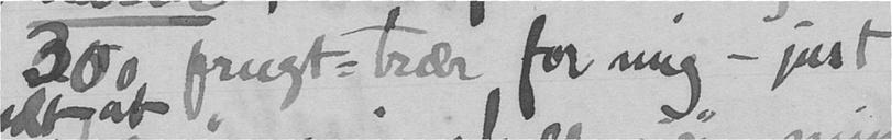300 frugt-trær for mig - just
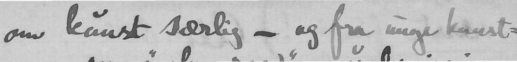om kunst særlig - og fra unge kunst-
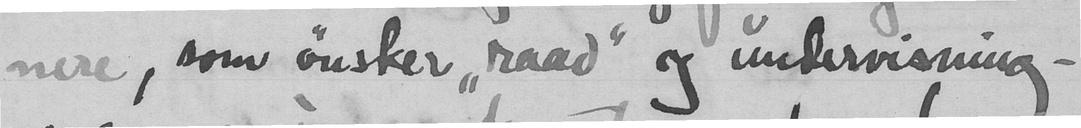nere, som ønsker "raad" og undervisning -
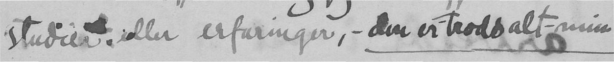studier, eller erfaringer, - den er - trods alt - min
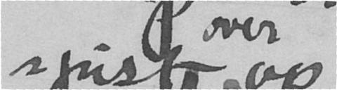spist op
